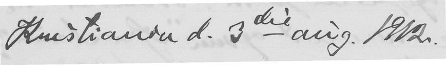Kristiania d. 3die aug. 1912.
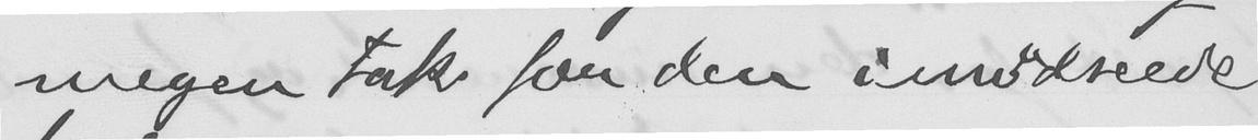megen tak for den imødseede
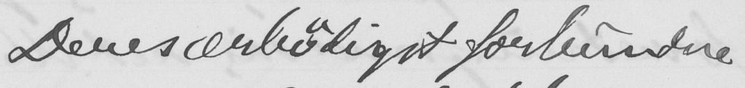Deres ærbødigst forbundne
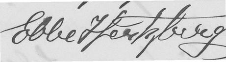Ebbe Hertzberg
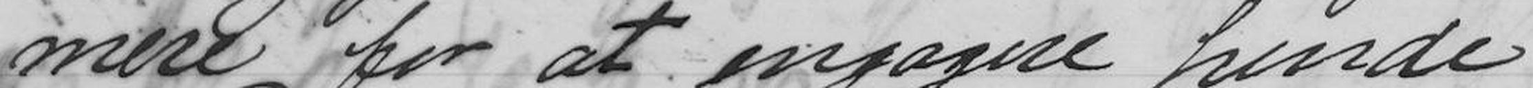mere for at engagere hende
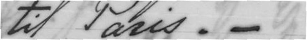til Paris. -
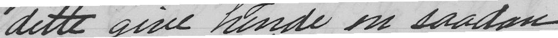dette give hende en saadan
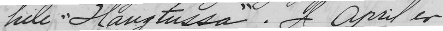hele "Haugtussa". I April er
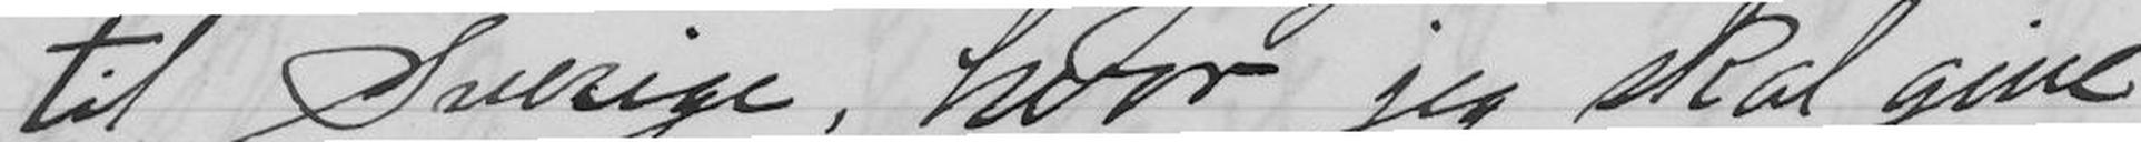til Sverige, hvor jeg skal give
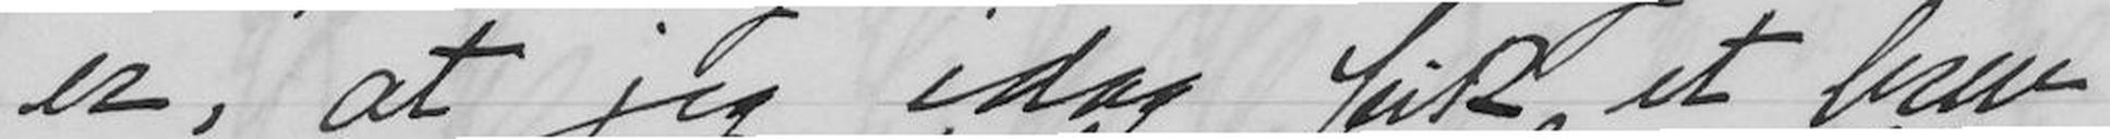er, at jeg idag fik et brev
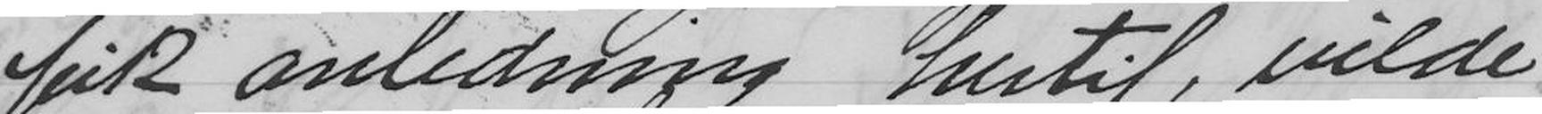fik anledning hertil, vilde
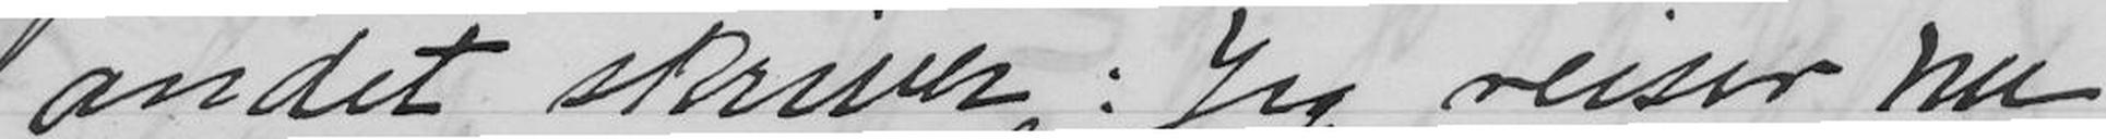andet skriver: Jeg reiser nu
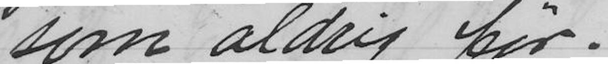som aldrig før.
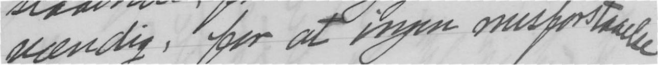vændig, for at ingen misforstaaelse
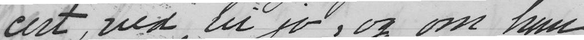cert, ved vi jo, og om hun
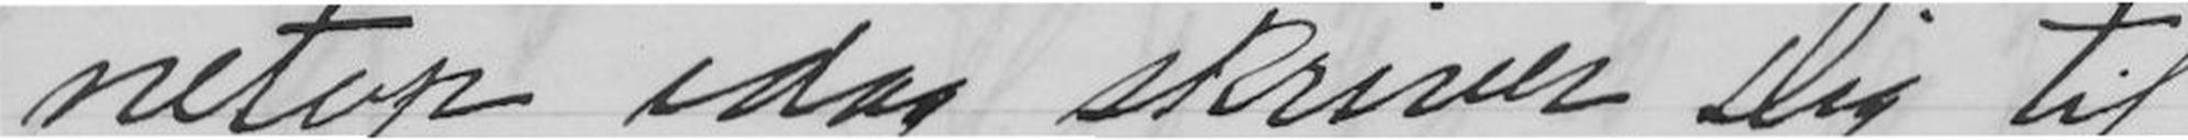netop idag skriver Dig til
